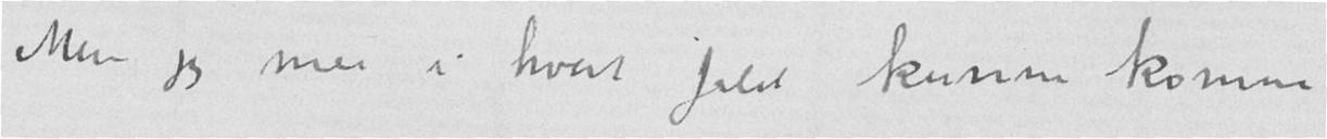Men jeg maa i hvert fald kunne komme
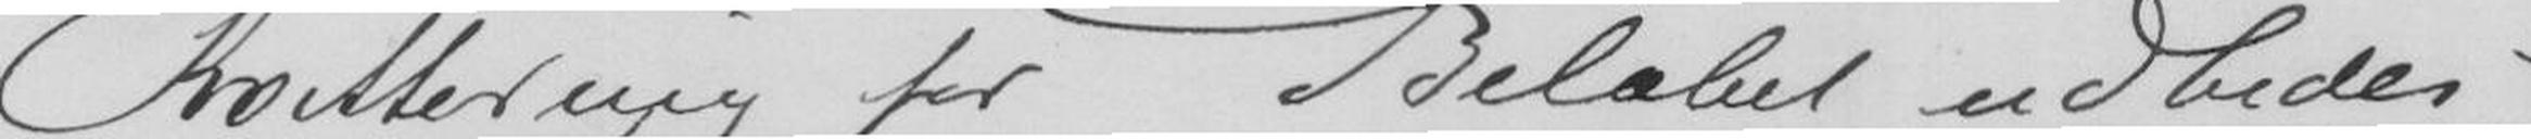Kvittering for Beløbet udbedes -
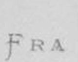FRA
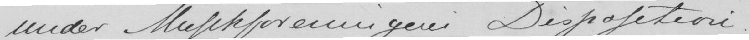under Musikforeningens Disposition.
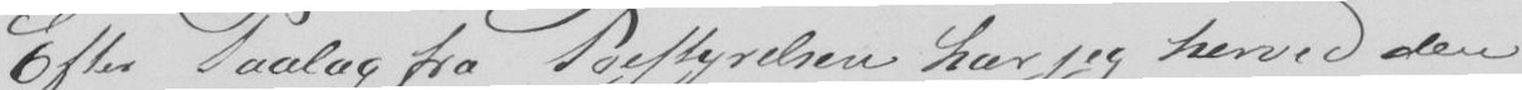Efter Paalæg fra Bestyrelsen har jeg herved den
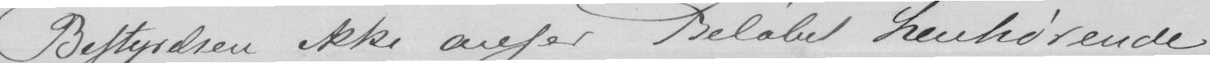Bestyrelsen ikke anser Beløbet herhørende
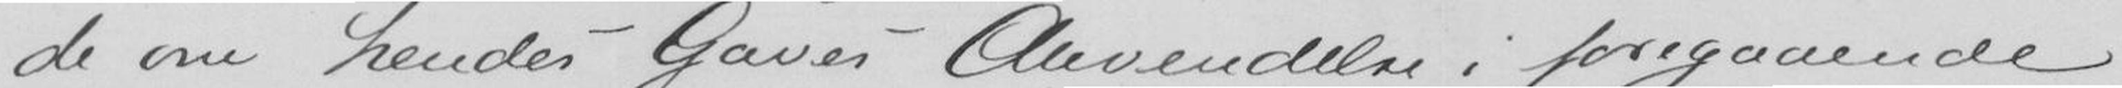de om hendes Gaver Anvendelse i foregaaende
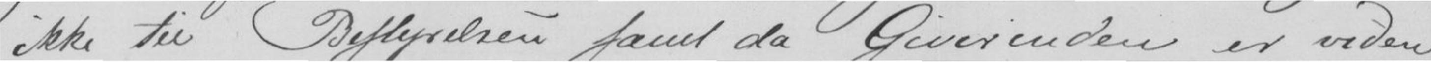ikke til Bestyrelsen fant da Giverinden er viden-
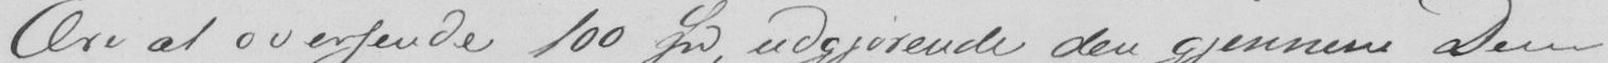Ære at oversende 100 Spd, udgjørende den gjennem Dem
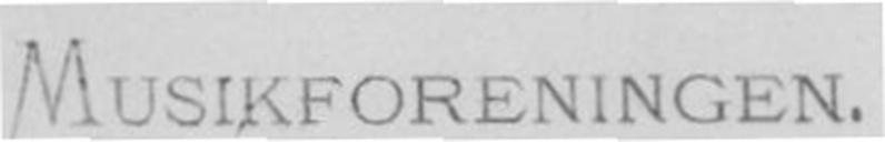MUSIKFORENINGEN.
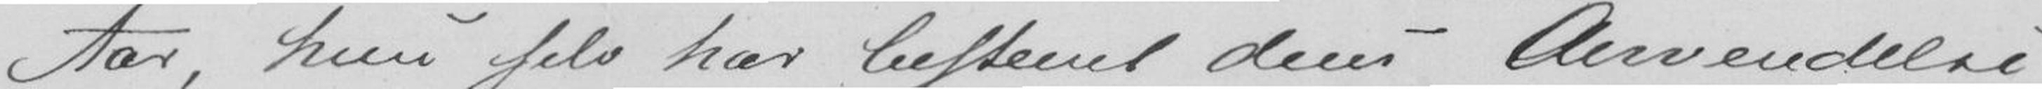Aar, hun selv har bestemt dens Anvendelse
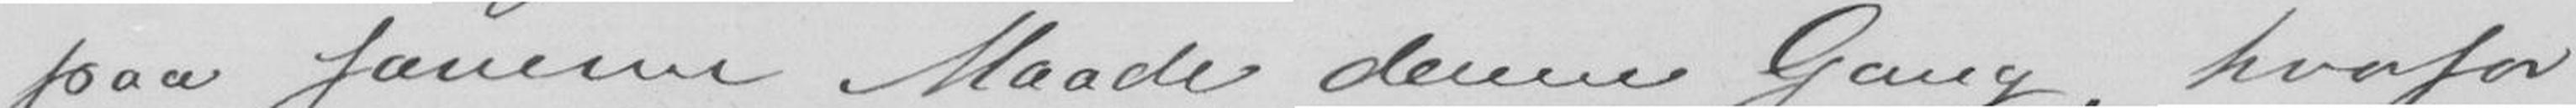paa samme Maade denne Gang, hvorfor
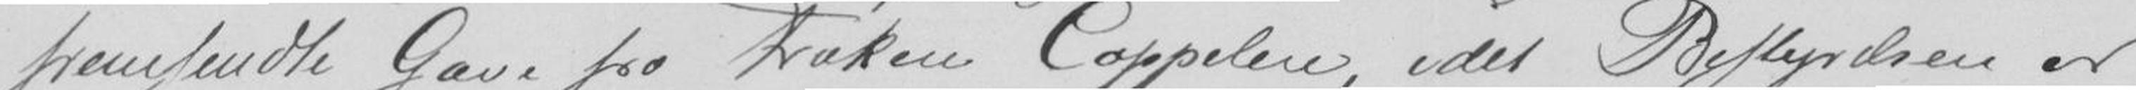fremsendte Gave fra Frøken Cappelen, idet Bestyrelsen er
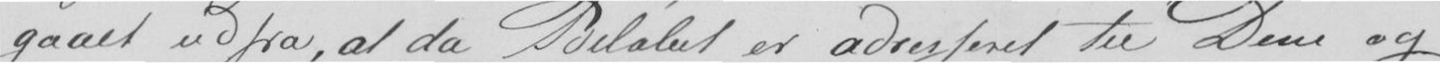gaaet udfra, at da Beløbet er adresseret til Dem og
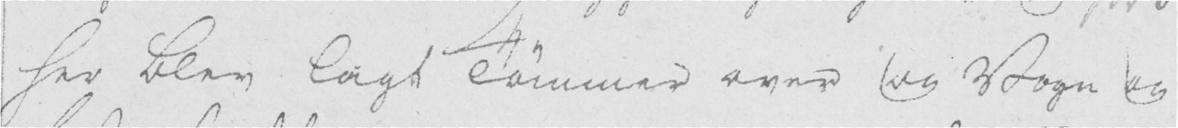her blev lagt Tømmer over og Vogn og
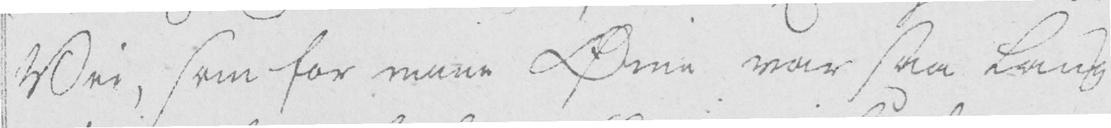Vei, som for mine Øine var saa Lang
